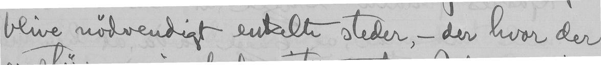blive nødvendigt enkelte steder, - der hvor der
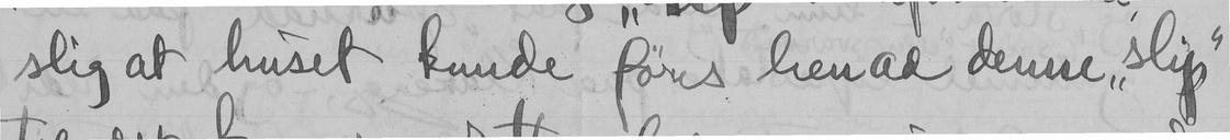slig at huset kunde føres henad denne "slip"
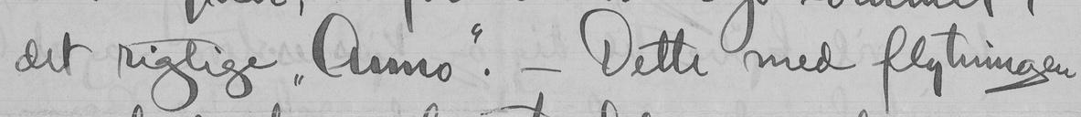det rigtige "Anno". - Dette med flytningen
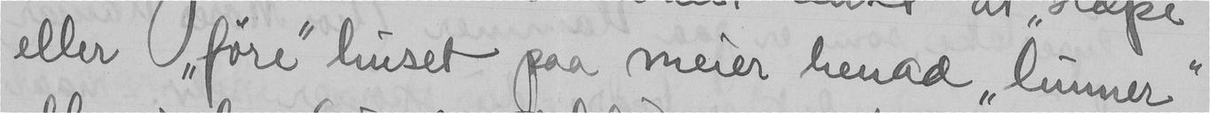eller i "føre" huset paa meier henad "lunner"
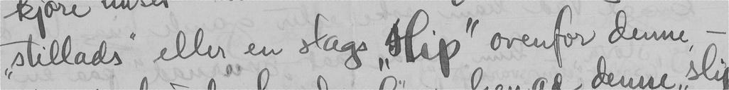"stillads" eller en "slip" ovenfor denne, -
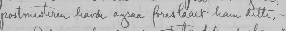postmesteren havde ogsaa foreslaaet ham dette, -
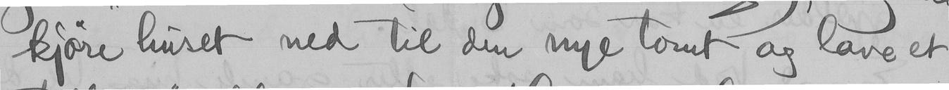kjøre huset ned til den nye tomt og lave et
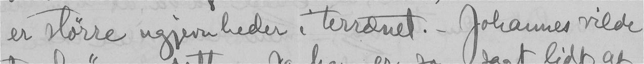er større ugjevnheder i terrænet. - Johannes vilde
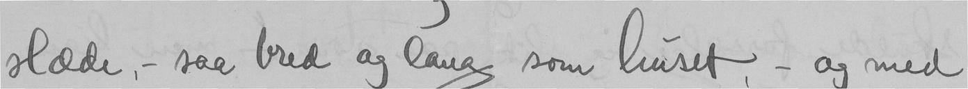slæde, - saa bred og lang som huset, - og med
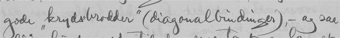gode "kryds-brodder" (diagonalbindinger), - og saa
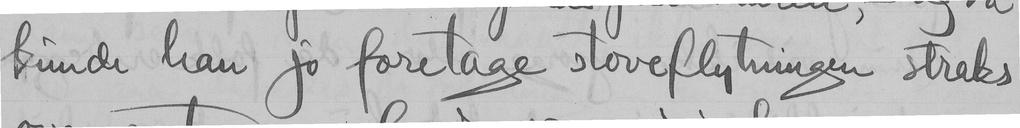kunde han jo foretage stoveflytningen straks
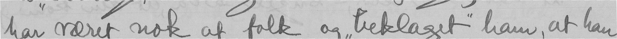har været nok af folk og "beklaget" ham, at han
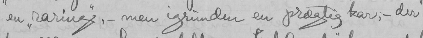en "raring", - men igrunden en prægtig kar; - der
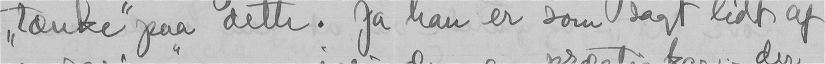"tænke" paa dette. Ja han er som sagt lidt af
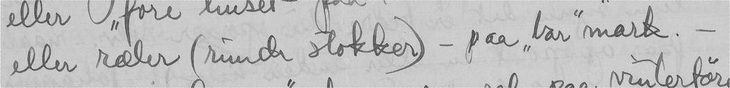eller ræler (runde stokker) - paa "bar" mark. -
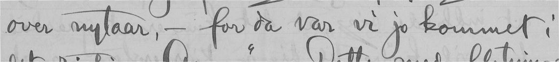over nytaar, - for da var vi jo kommet i
